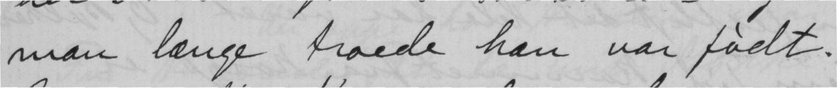man længe troede han var født.
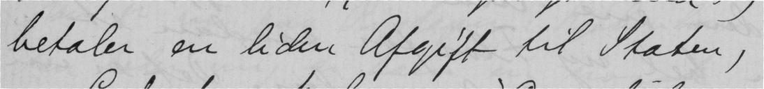betaler en liden Afgift til Staten,
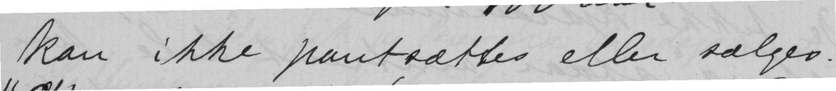kan ikke pantsættes eller sælges.
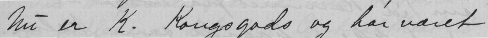Nu er K. Kongsgods og har været
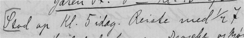Stod op Kl. 5 idag. Reiste med 1/2 7
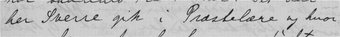her Sverre gik i Præstelære og hvor
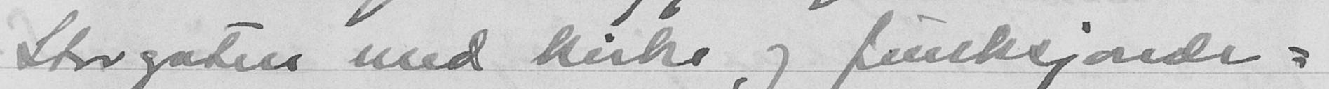Storgaten med kirke og funksjønde-
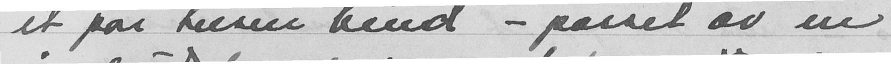et par tusen bind - passet av en
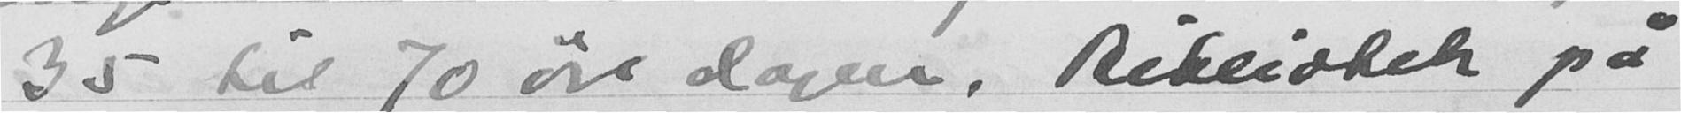35 til 70 øre dagen, Bibliotek på
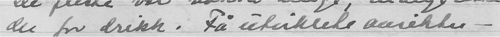der for drikk. Få utviklede ansikter -
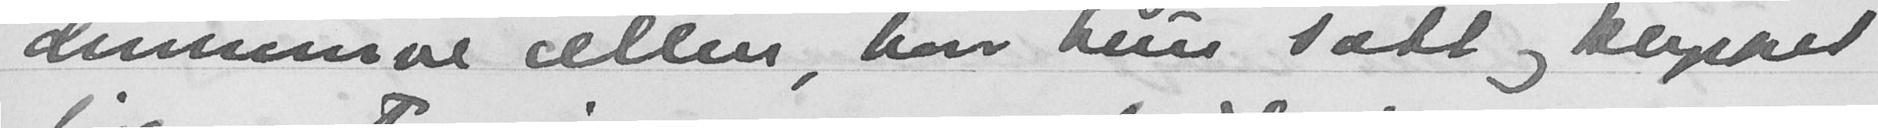diminuve cellen, hvor hun satt og klippet
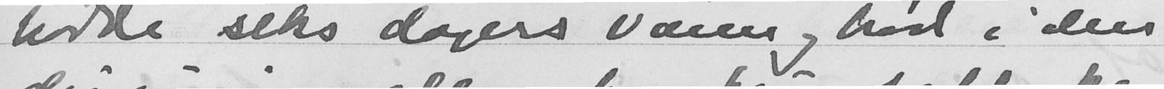hadde seks dagers vann og brød i den
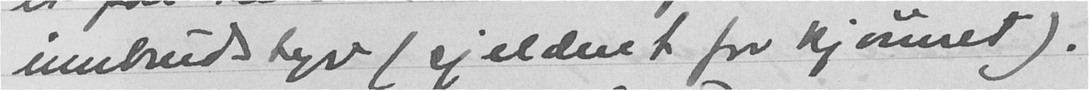innbrudstyv (sjeldent for kjønnet).
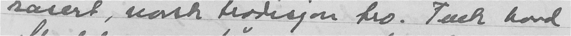rasert, norsk tradisjon tro. Tenk hvad
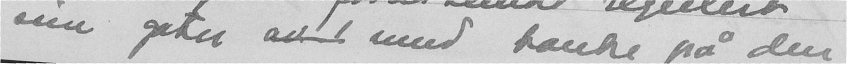sine gater  med banke på den
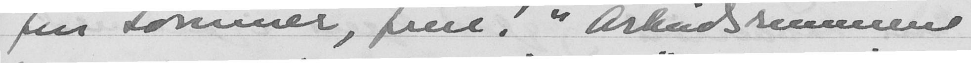fin sommer, frue. " Orkidesummene
